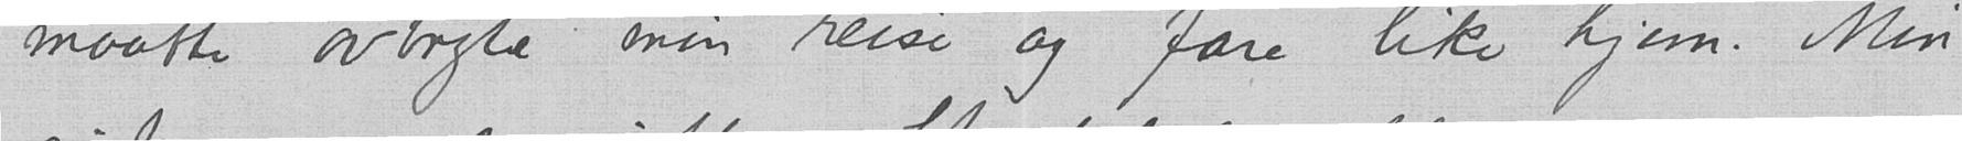maatte avbryte min reise og fare like hjem. Min
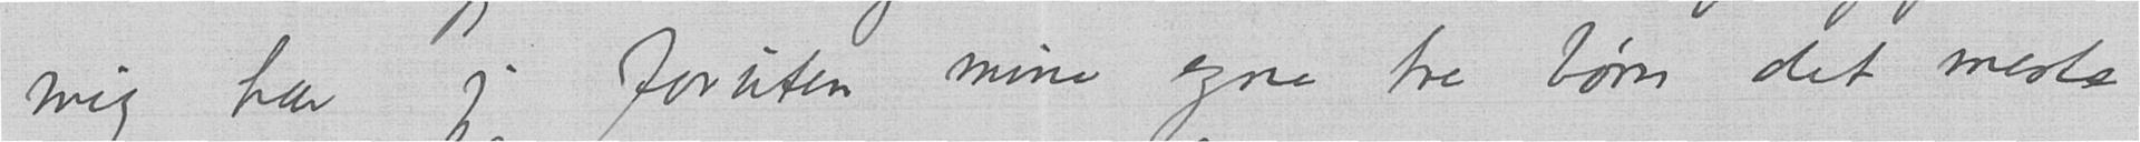mig har jeg foruten mine egne tre børn det meste
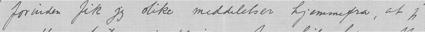forinden fik jeg slike meddelelser hjemmefra, at jeg
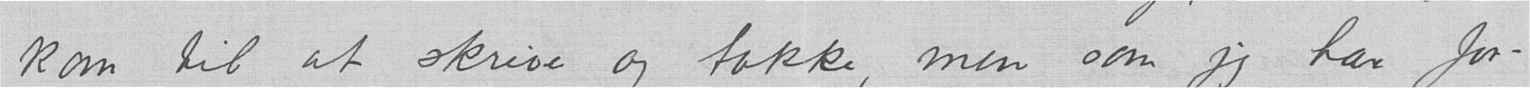kom til at skrive og takke, men som jeg har for-
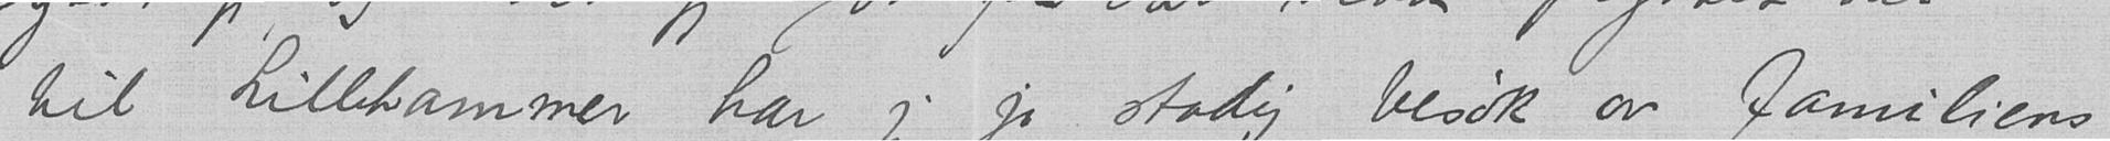til Lillehammer har jeg jo stadig besøk av familiens
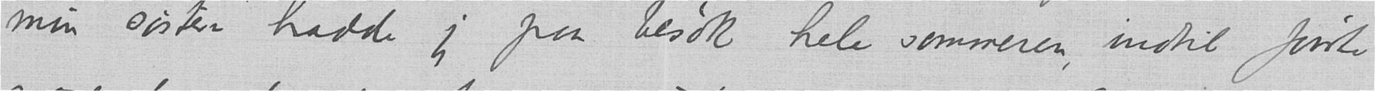min søster hadde jeg paa besøk hele sommeren, indtil første
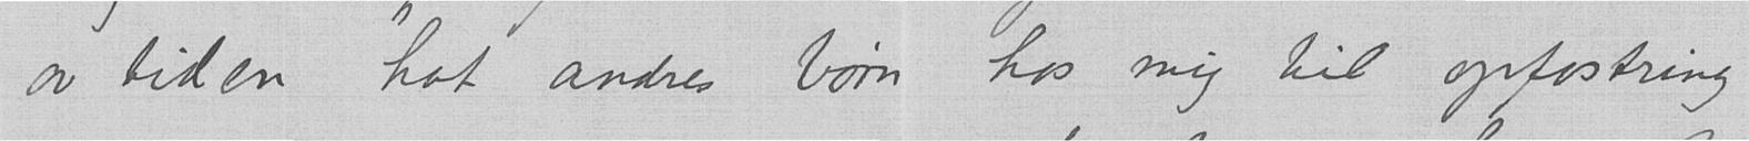av tiden hat andres børn hos mig til opfostring
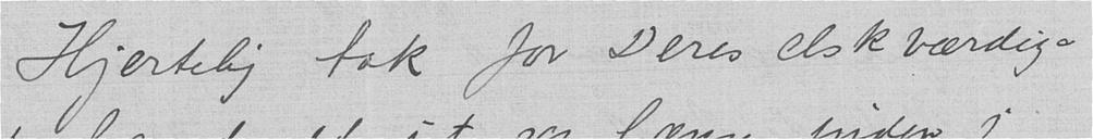Hjertelig tak for Deres elskværdige
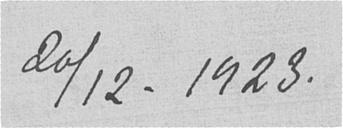20/12-1923.
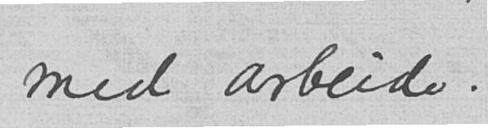med arbeide.
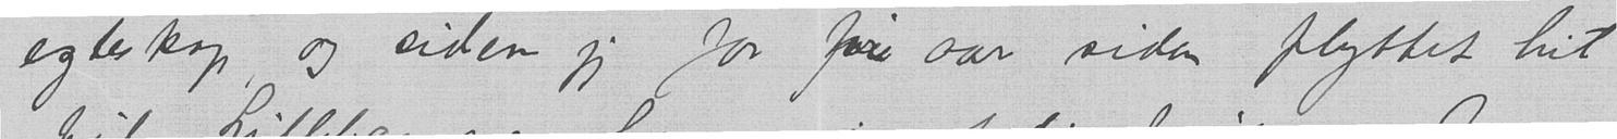egteskap, og siden jeg for fire aar siden flyttet hit
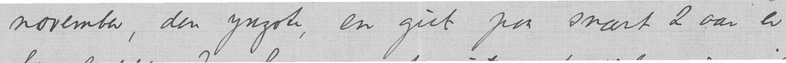november, den yngste, en gut paa snart 2 aar er
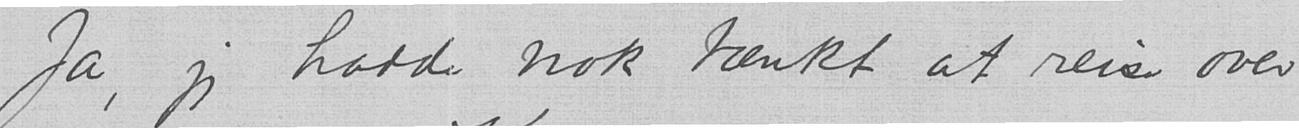Ja, jeg hadde nok tænkt at reise over
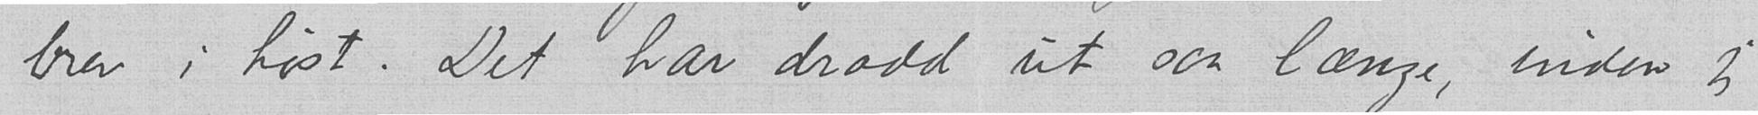brev i høst. Det har dradd ut saa længe, inden jeg
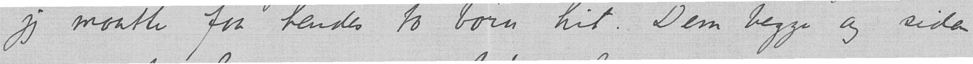jeg maatte faa hendes to børn hit. Dem begge og siden
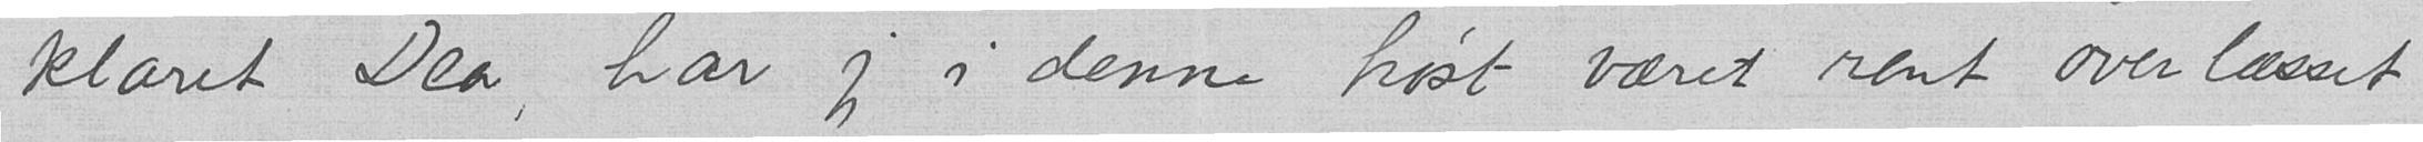klaret Dea, har jeg i denne høst været rent overlæsset
